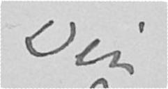Din
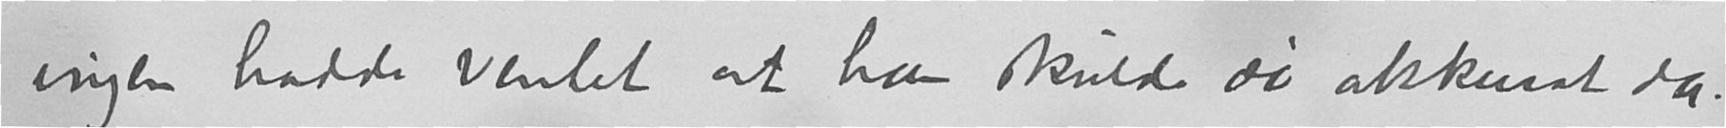ingen hadde ventet at han skulde dø akkurat da.
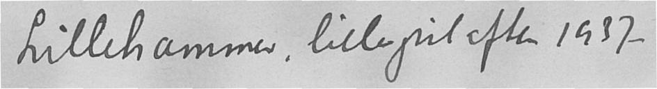Lillehammer, lillejuilaften 1937
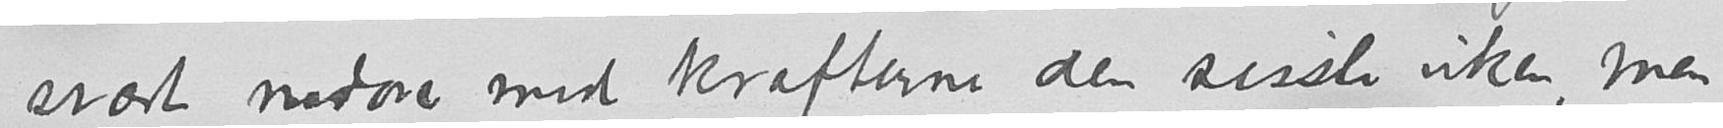svært nedover med kræfterne den sisste uken, men
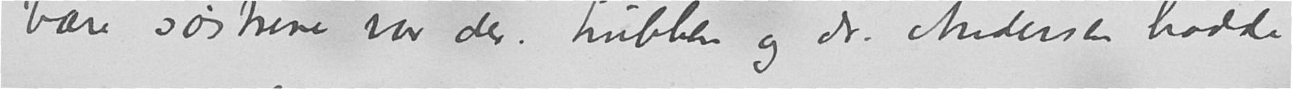bare søstrene var der. Lubben og dr. Andersen hadde
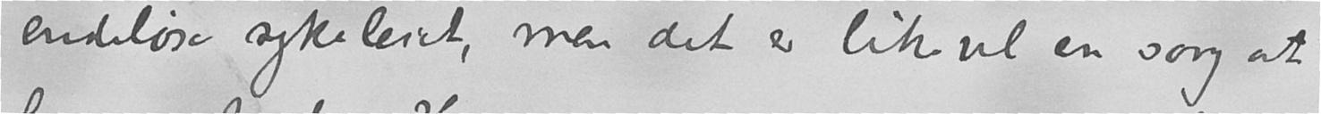endeløse sykeleiet, men det er likevel en sorg at
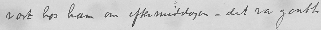vært hos ham om eftermiddagen – det var gaatt
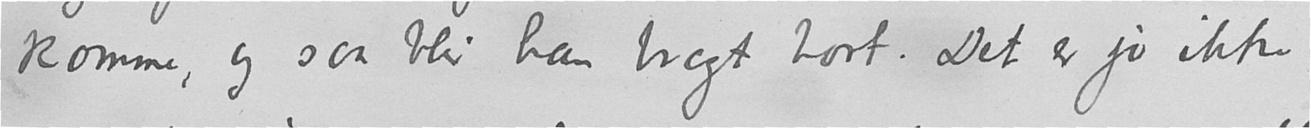komme, og saa blir han bragt bort. Det er jo ikke
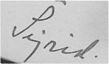Sigrid.
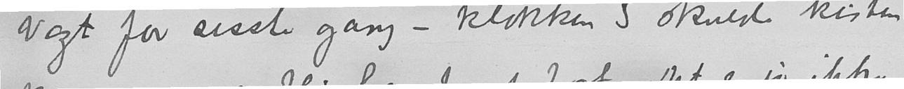Vogt for siste gang – klokken 3 skulde kisten
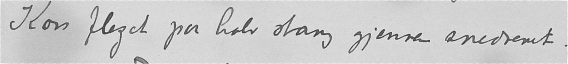Kors flaget paa halv stang gjennem snedrenet.
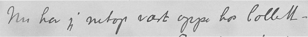Nu har jeg netop vært oppe hos Collett -
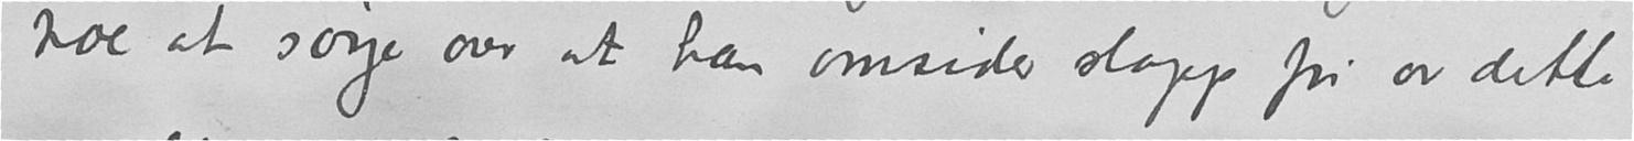noe at sørge over at han omsider slapp fri av dette
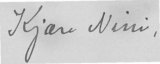Kjære Nini,
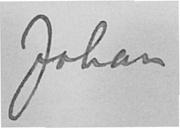Johan
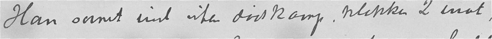Han sovnet ind uten dødskamp, klokken 2 inat
